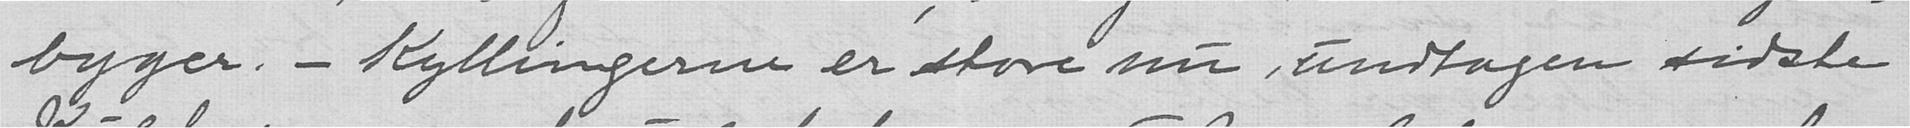byger. - Kyllingerne er store nu, undtagen sidste
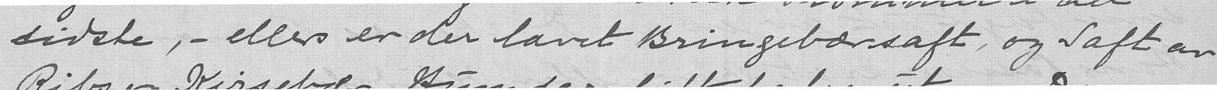sidste, - ellers er der lavet Bringebærsaft, og Saft av
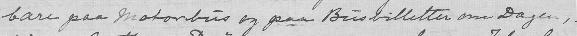bare paa Motorbus og paa Busbilletter om Dagen, -
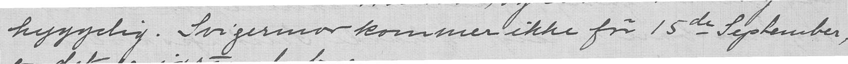hyggelig. Svigermor kommer ikke før 15de September,
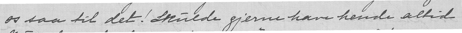os saa til det! Skulde gjerne have hende altid.
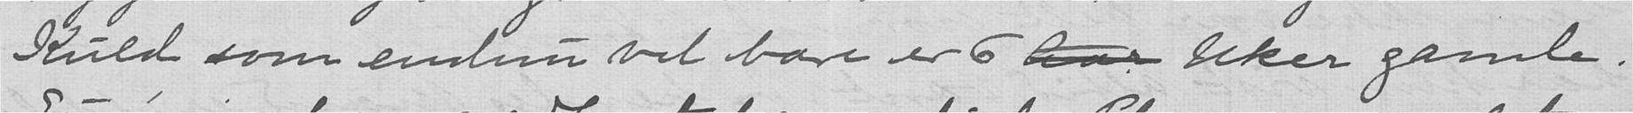Kuld som endnu vel bare er 6  Uker gamle.
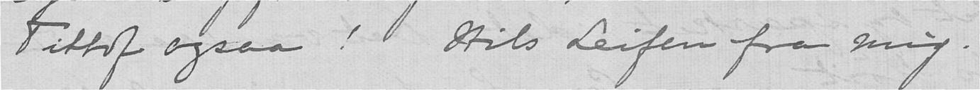Tittof ogsaa! Hils Leifen fra mig.
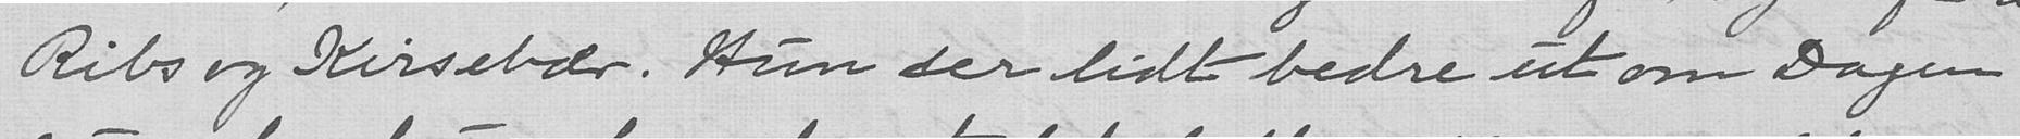Ribs og Kirsebær. Hun ser lidt bedre ut om Dagen
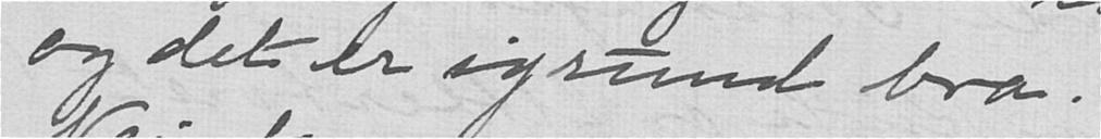og det er igrund bra.
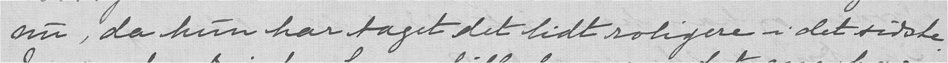nu, da hun har taget det lidt roligere i det sidste.
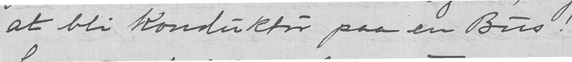at bli Konduktør paa en Bus!
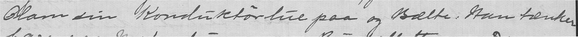Olam sin Konduktørlue paa og Bælte. Han tænker
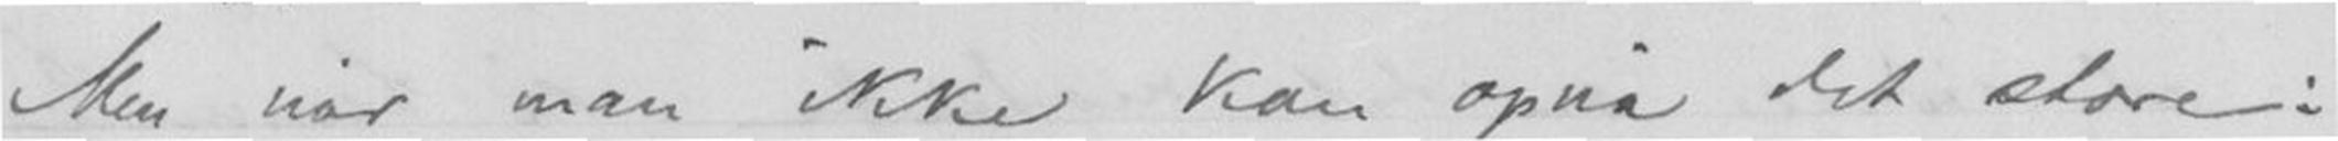Men når man ikke kan opnå det store:
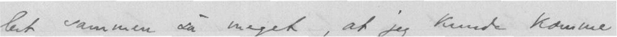bet sammen så meget, at jeg kunde komme
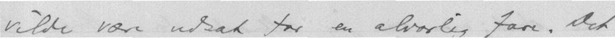vilde være udsat for en alvorlig fare. Det
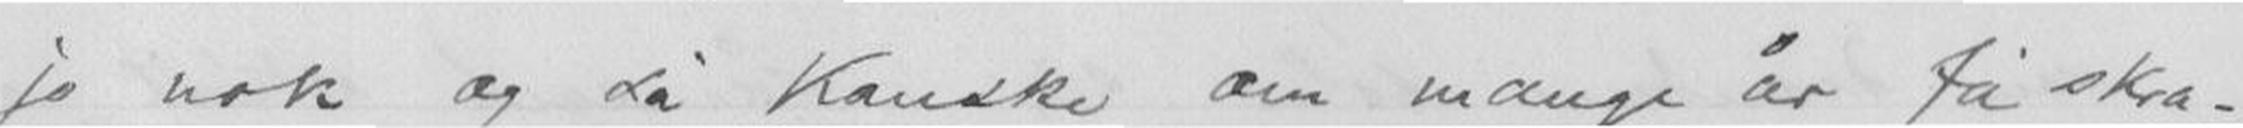jo nok og då kanske om mange år få skra-
